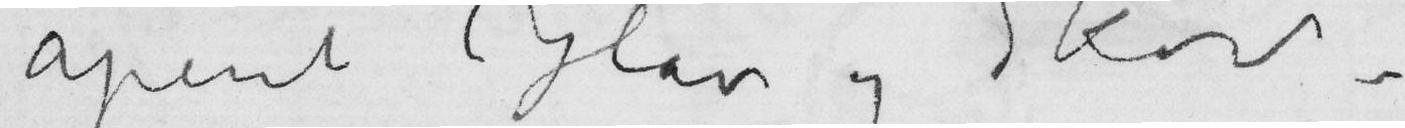åpent Hav og Skov –
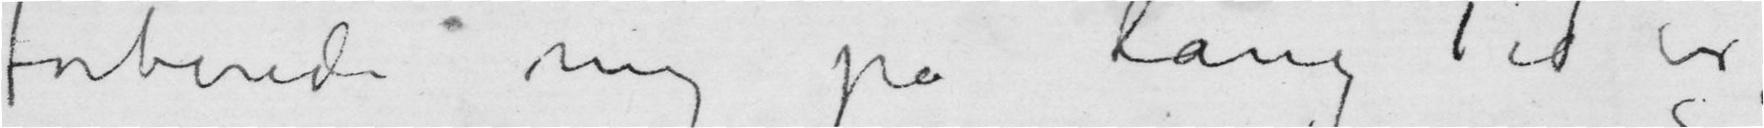forberede mig på lang Tid
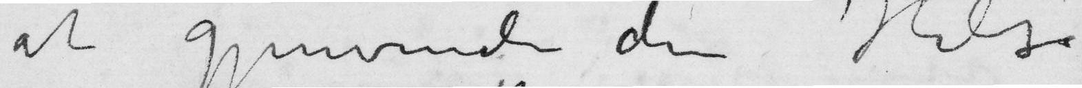at gjenvinde den Helse
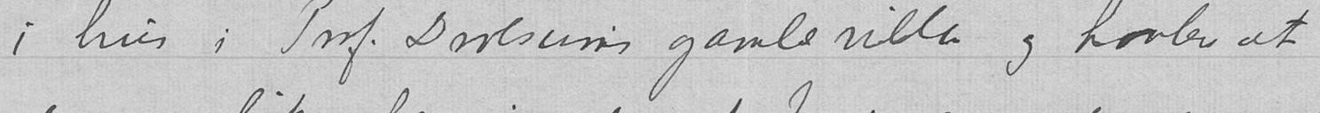i hus i Prof. Drolsums gamle villa og haaber at
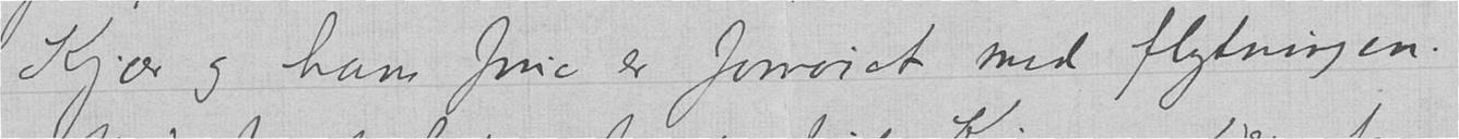Kjær og hans frue er fornøiet med flytningen.
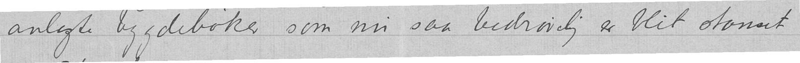anlagte bygdebøker, som nu saa bedrøvelig er blit stanset
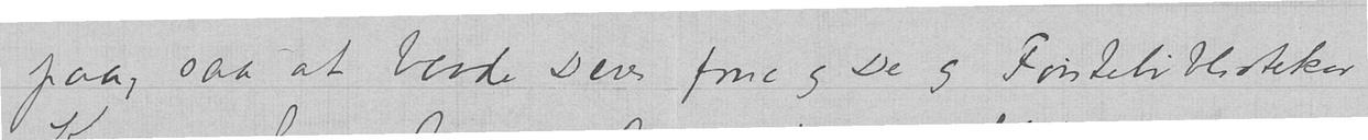paa, saa at baade Deres frue og De og Førstebibliotekar
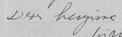Deres hengivne
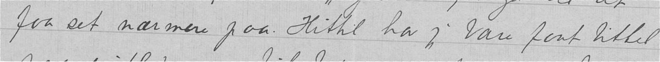faa set nærmere paa. Hittil har jeg bare faat tittet
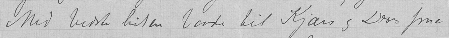Med bedste hilsen baade til Kjærs og Deres frue
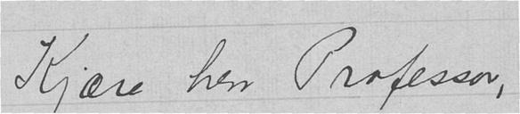Kjære herr Professor,
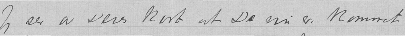Jeg ser av Deres kort at De nu er kommet
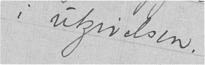i utgivelsen.
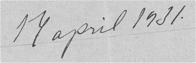17 april 1931.
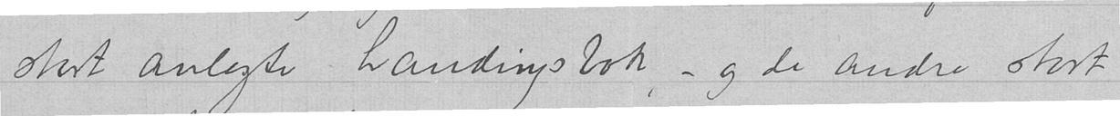stort anlagte Landingsbok, - og de andre stort
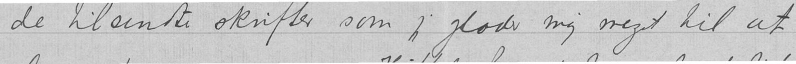de tilsendte skrifter som jeg glæder mig meget til at
